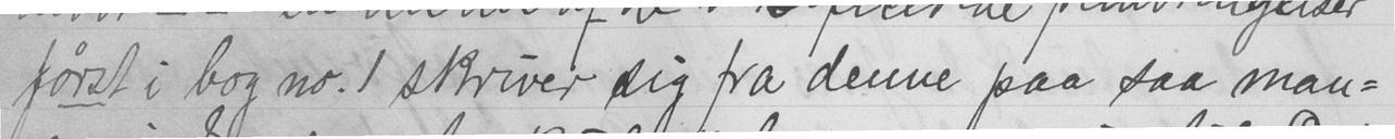først i bog no. 1 skriver sig fra denne paa saa man-
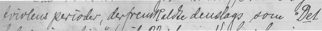tvivlens perioder, derfremkaldte denslags som "Det
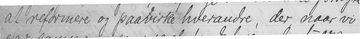at reformere og paavirke hverandre, der, naar vi
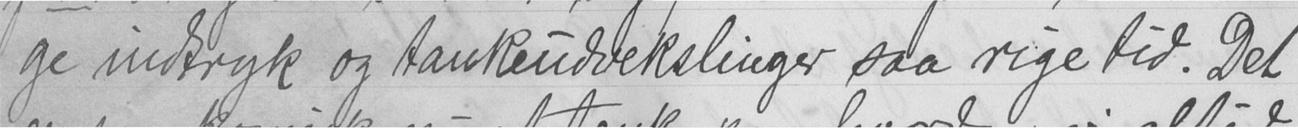ge indtryk og tankeudvekslinger saa rige tid. Det
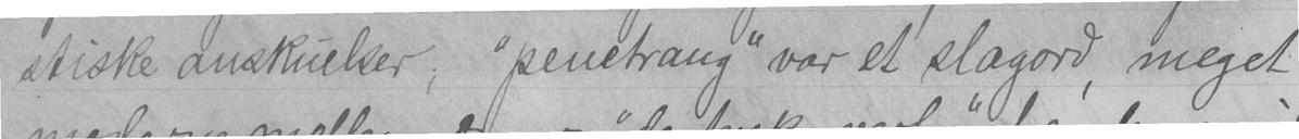stiske anskuelser; "penetrang" var et slagord, meget
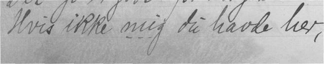Hvis ikke mig du havde her,
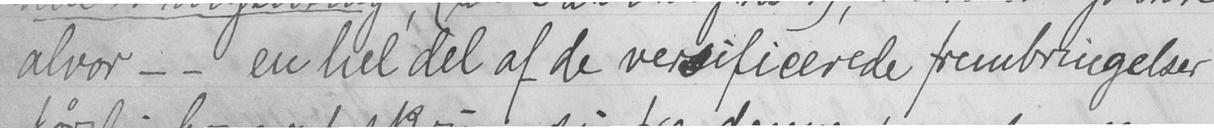alvor - - en hel del af de versificerede frembringelser
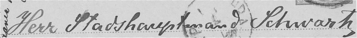Herr Stadshauptmand Schwartz
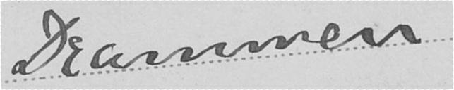Drammen
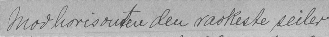Mod horisonten den raskeste seiler
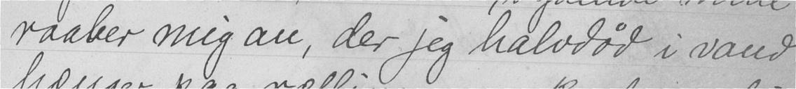raaber mig an, der jeg halvdød i vand
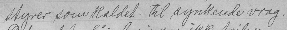styrer som kaldet til synkende vrag.
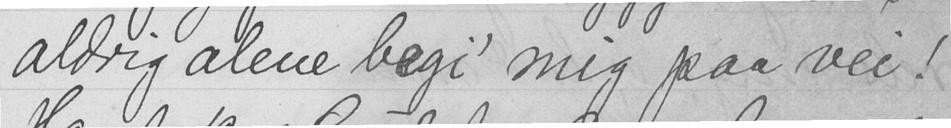aldrig alene begi' mig paa vei!
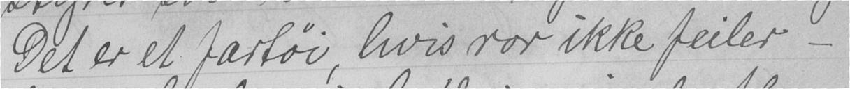Det er et fartøi, hvis ror ikke feiler -
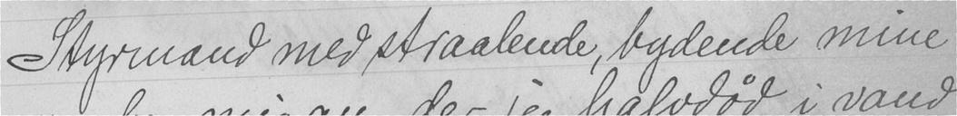Styrmand med straalende, bydende mine
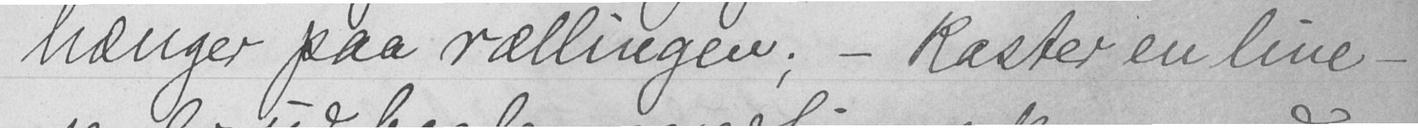hænger paa rællingen; - kaster en line -
